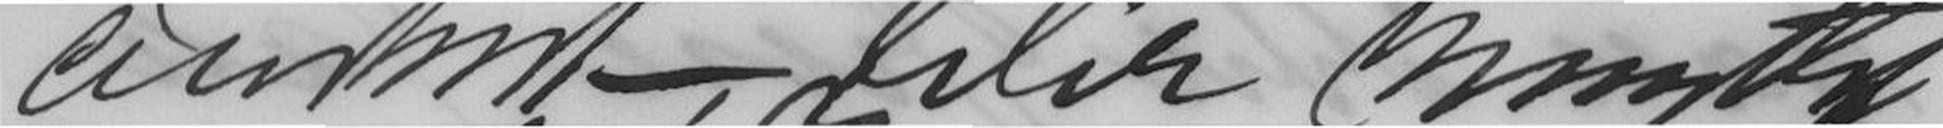ønsket, blir knyttet
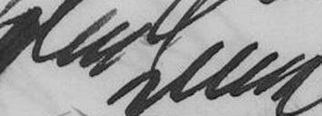John Lund
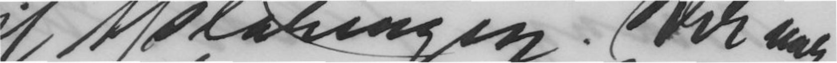til Afsløringen. Der var
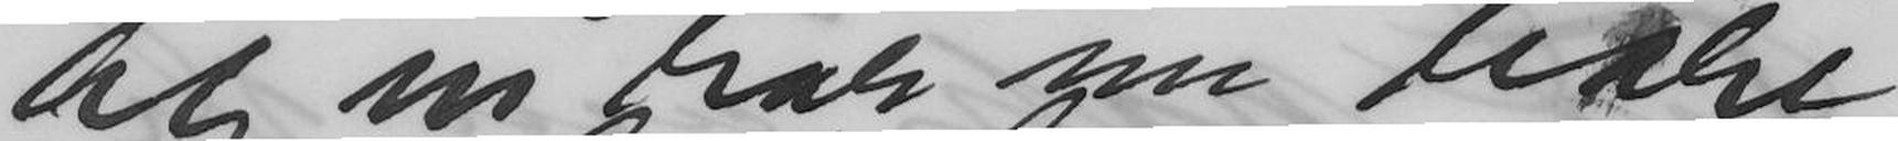og vi har nu bare
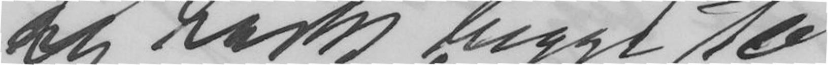og rask begge to
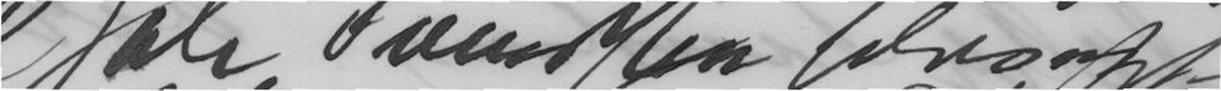Joh Svendsen selvsagt
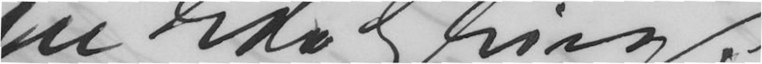en Edv Grieg.
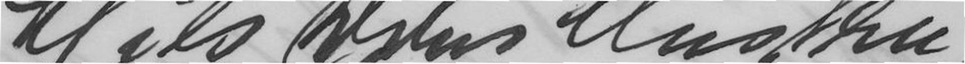Hils Deres Hustru
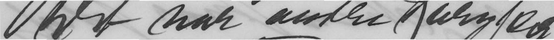Det var andre Kuryfeer
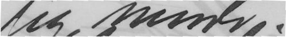jeg mente.
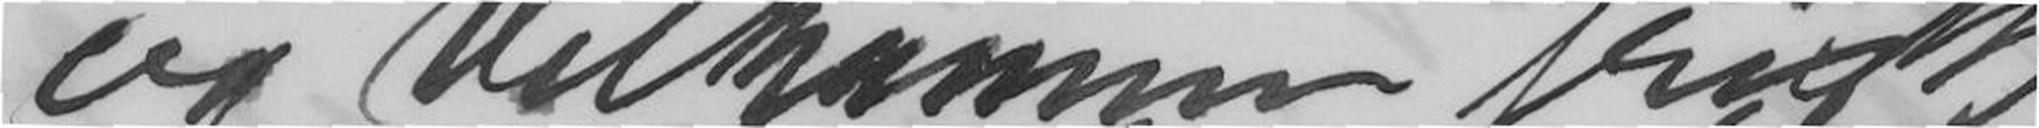og Velkommen frisk
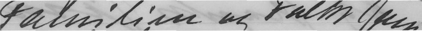Familien og Folk som
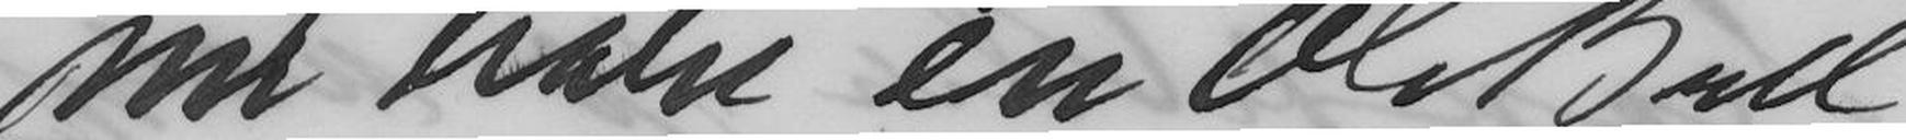nu bare en Ole Bull
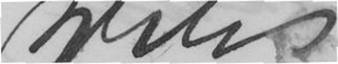Deres
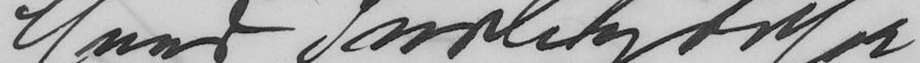Hvad Indbydelser
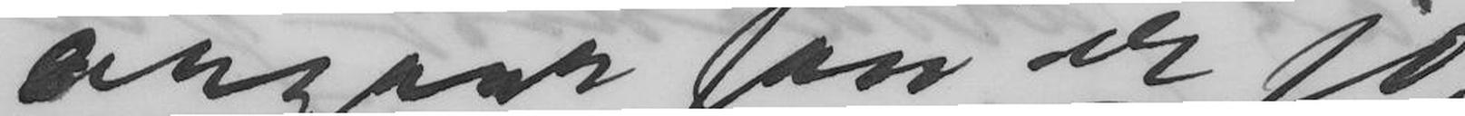angaar saa er jo
